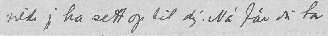vilde jeg ha sett op til dig. Nå får du ta
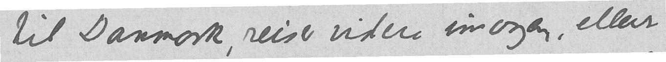til Danmark, reiser videre imorgen, ellers
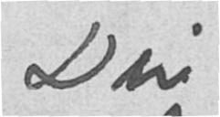Din
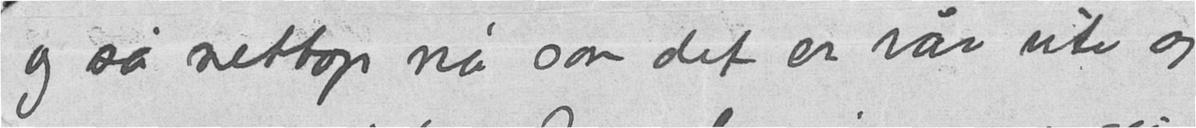og så nettop nå som det er vår ute og
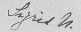Sigrid U.
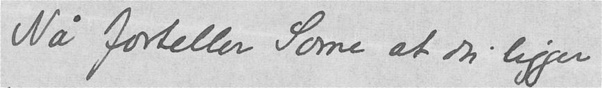Nå forteller Sorne at du ligger
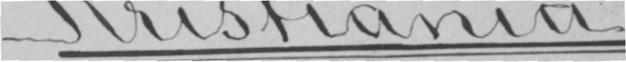Kristiania.
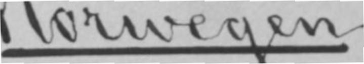Norwegen.
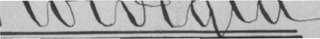(Norvegia.)
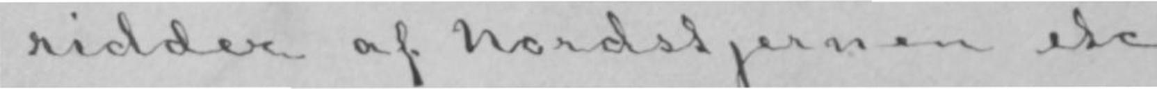ridder af Nordstjernen etc.
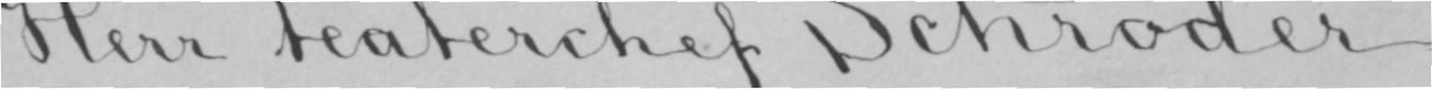Herr teaterchef Schrøder,
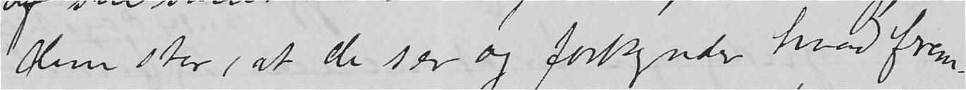dem stor, at de ser og forkynder hvad frem-
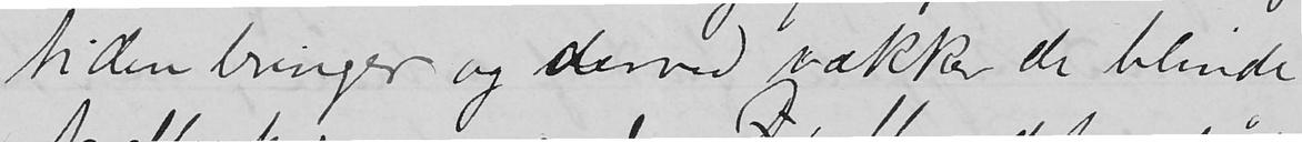tiden bringer og derved vækker de blinde
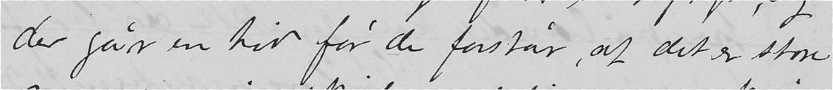der går en tid før de forstår, at det er store
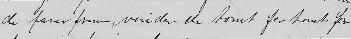de farer frem, vinder de tomt for tomt for
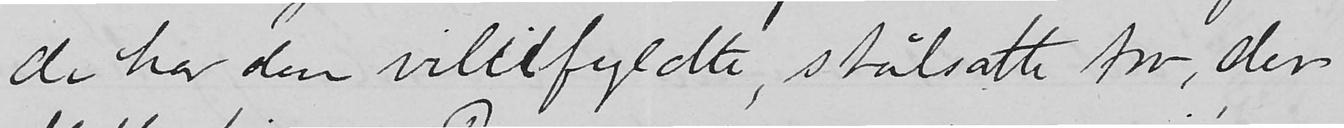de har den viliefyldte, stålsatte tro, der
